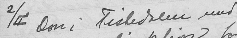2/II Don i Tistedalen med
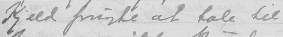Kjeld forsøgte at tale til
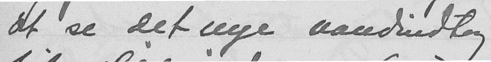at se det nye vandindtag
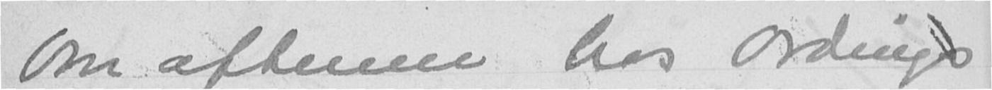Om aftenen hos Ordings.
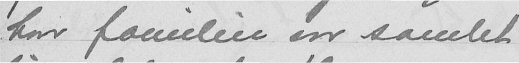hvor familien var samlet
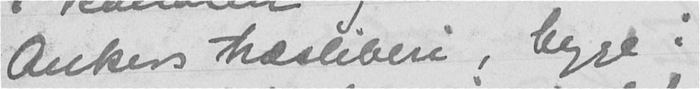Ankers træsliberi, begge i
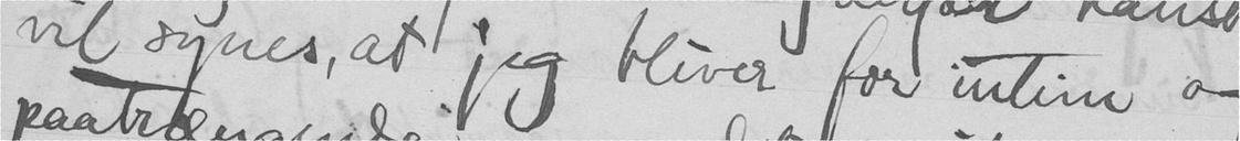vil synes, at jeg bliver for intim og
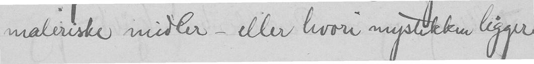maleriske midler - eller hvori mystikken ligger
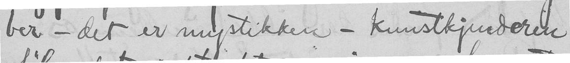ber; - det er mystikken - kunstkjenderen
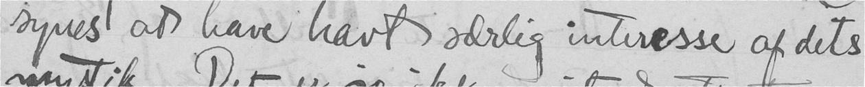synes at have havt særlig interesse af dets
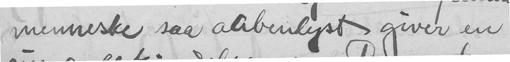menneske, saa abbenlyst giver en
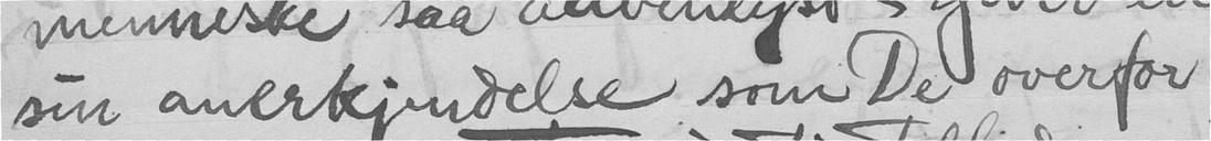sin anerkjendelse som De overfor
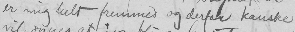er mig helt fremmed og derfor kanske
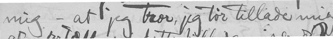mis - at jeg tror, jeg tør tillade mid
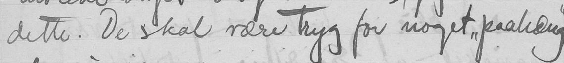dette. De skal være tryg for noget "paahæng"
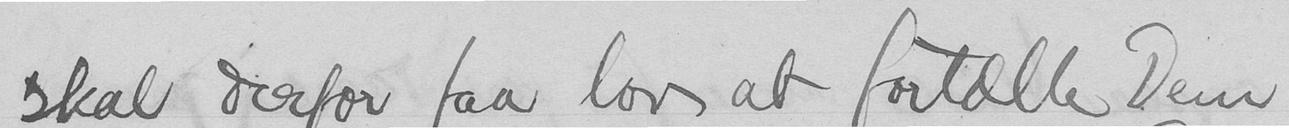skal derfor faa lov at fortælle Dem
